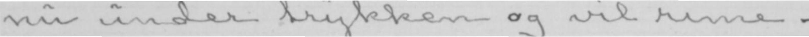nu under trykken og vil rime-
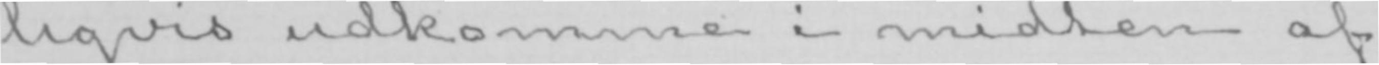ligvis udkomme i midten af
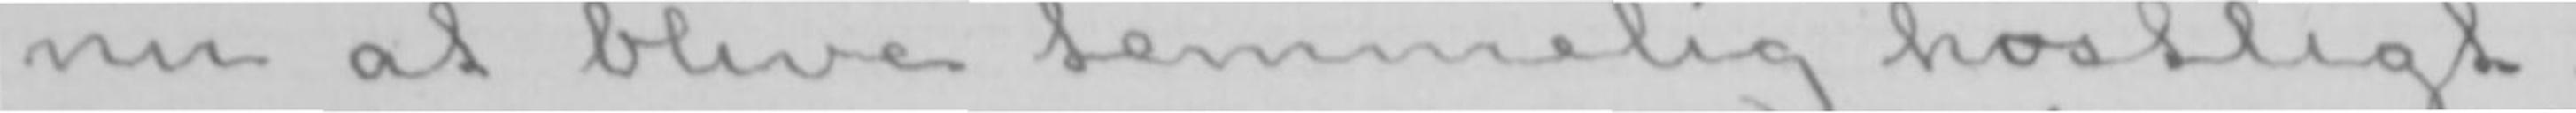nu at blive temmelig høstligt.
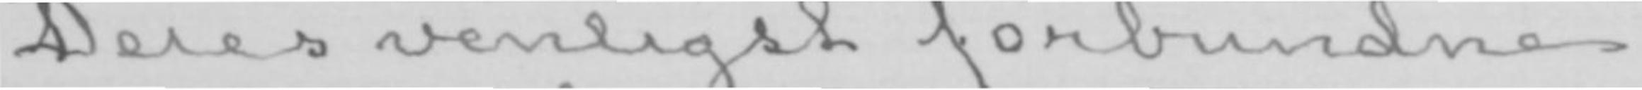Deres venligst forbundne
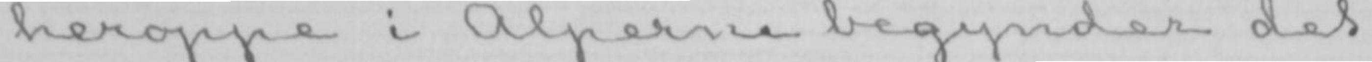heroppe i Alperne begynder det
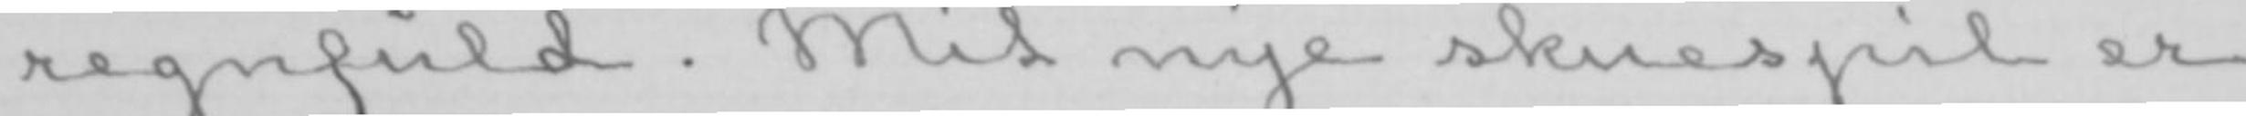regnfuld. Mit nye skuespil er
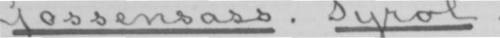Gossensass. Tyrol.
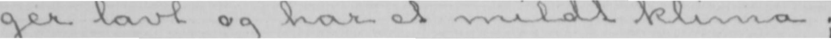ger lavt og har et mildt klima;
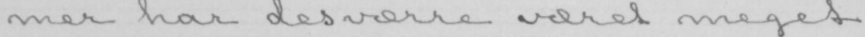mer har desværre været meget
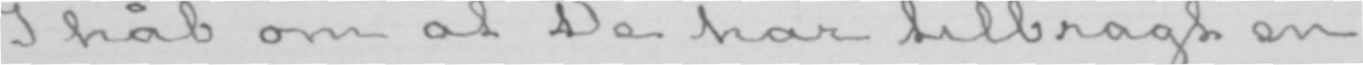I håb om at De har tilbragt en
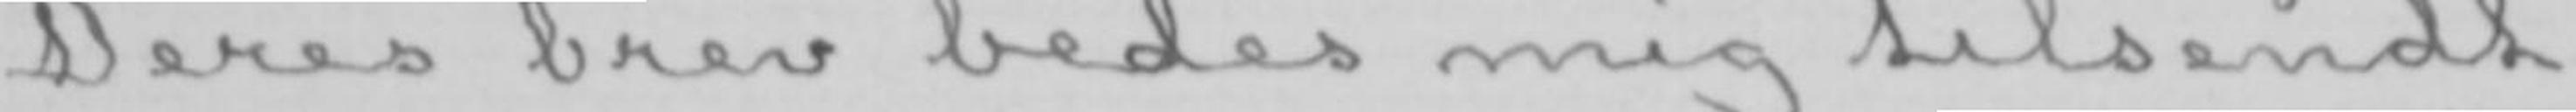Deres brev bedes mig tilsendt
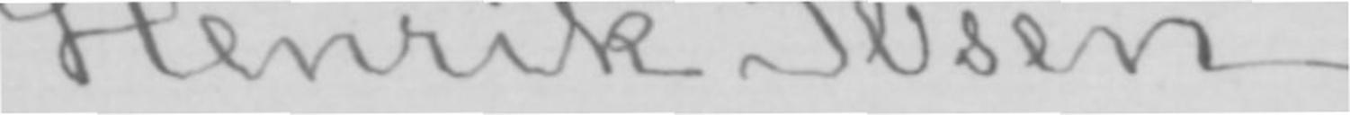Henrik Ibsen.
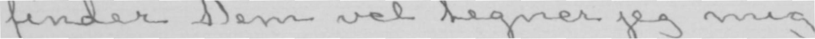finder Dem vel tegner jeg mig
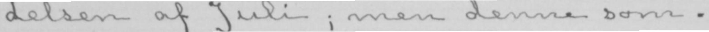delsen af Juli; men denne som-
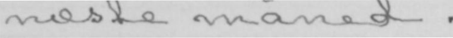næste måned.
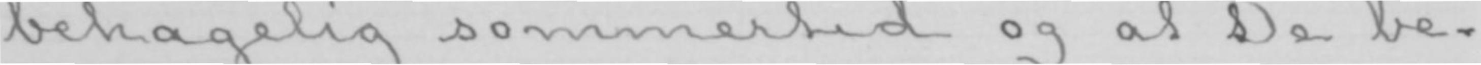behagelig sommertid og at De be-
